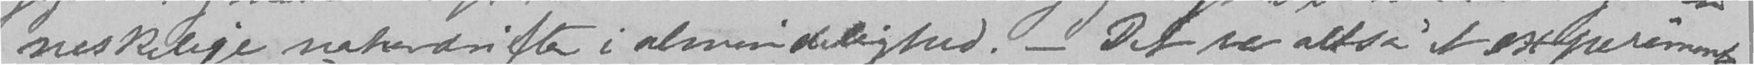neskelige naturdrifter i almindelighed! - Det var altså et experiment
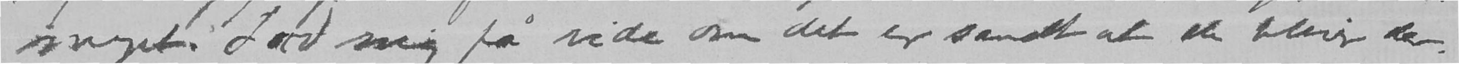noget. Lad mig få vide om det er sandt at de blir der
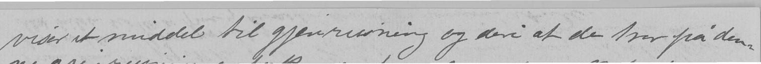viser et middel til gjenreisning og deri at de tror på den-
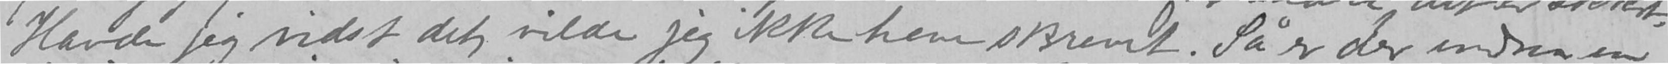Havde jeg vidst det, vilde jeg ikke have skrevet. Så er der endnu en
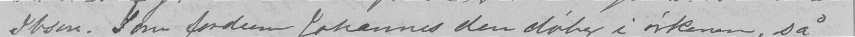Ibsen. Som fordum Johannes den døber i ørkenen, så
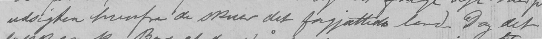udsigten hvorfra de skuer det forgjættede land. Dog det
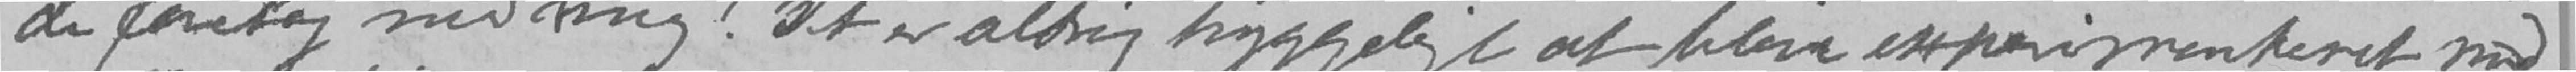de foretog med mig. Det er aldrig hyggeligt at blive exsperimenteret med
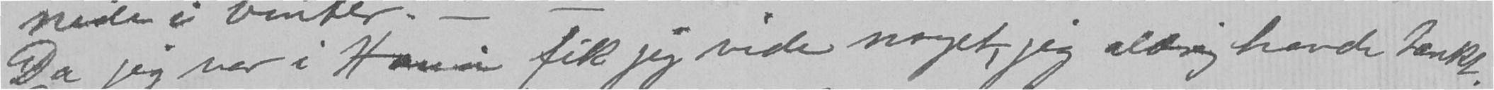Da jeg var i Xania fik jeg vide noget, jeg aldrig havde tænkt.
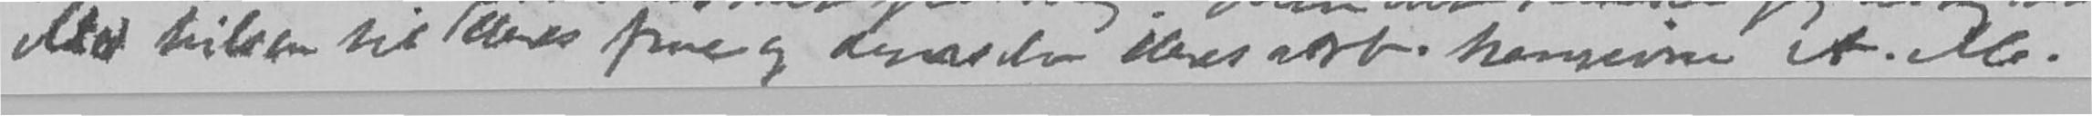Med hilsen til deres frue og dem selv deres hengivne A.M.
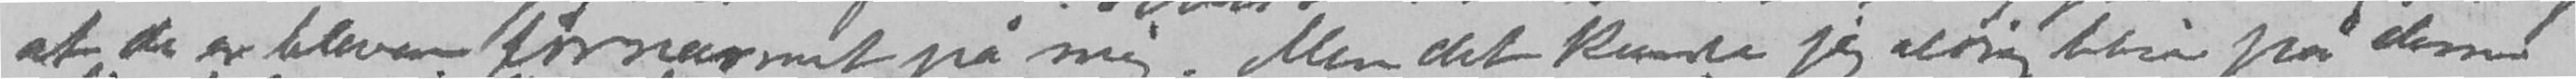at de er bleven fornærmet på mig. Men det kunde jeg aldri blive på dem.
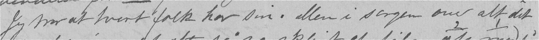Jeg tror at hvert folk har sin. Men i sorgen over alt, det
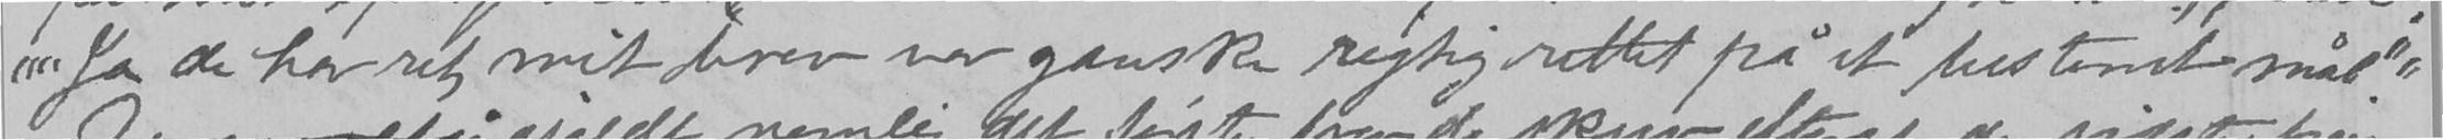"Ja de har ret, mit drev var ganske rigtig rettet på et bestemt mål".
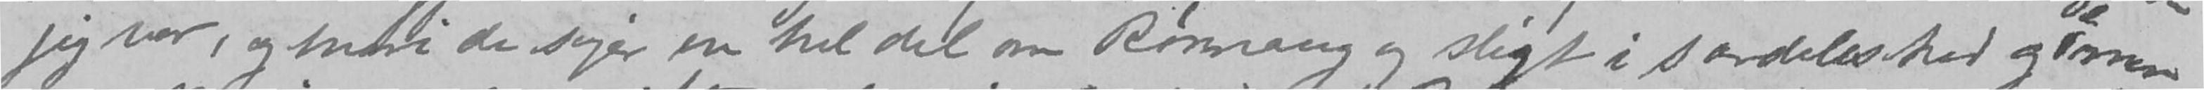jeg var, og hvori de siger en hel del om Rønnaug og sligt i særdeleshed og men-
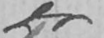er
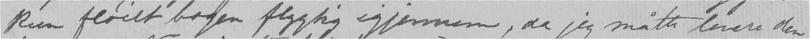kun fløiet bogen flygtig igjennem, da jeg måtte levere den
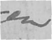en
